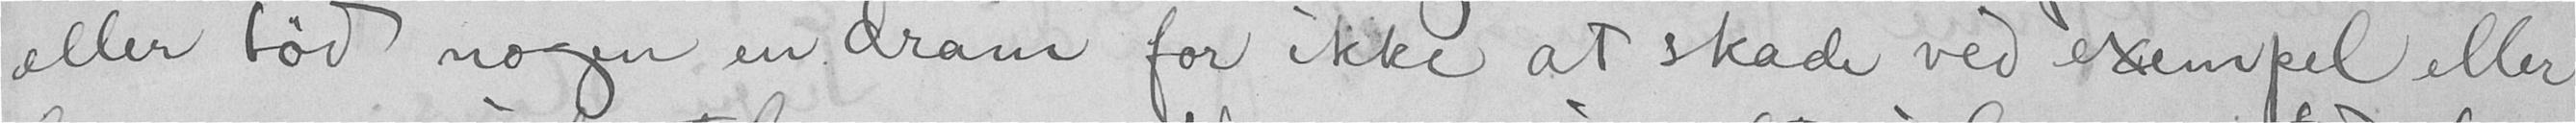eller tød nogen en dram for ikke at skade ved exempel eller
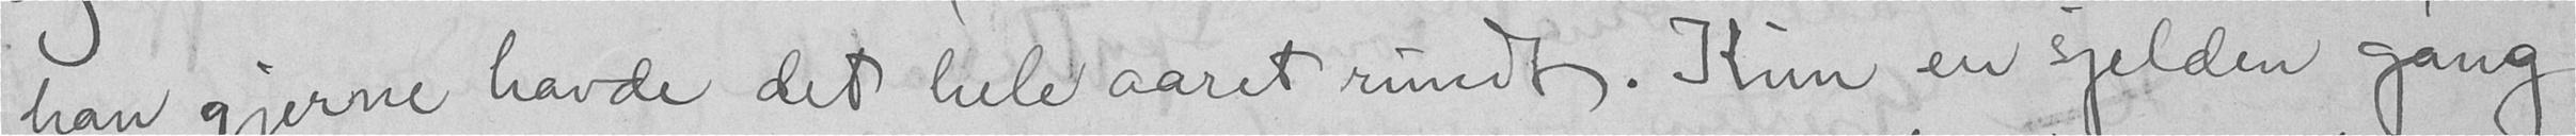han gjerne havde det hele aaret rundt. Kun en sjelden gang
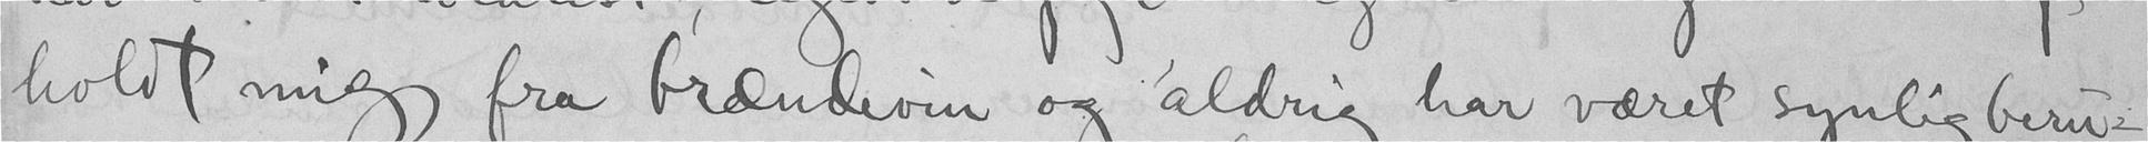holdt mig fra brændevin og aldrig har været synlig beru-
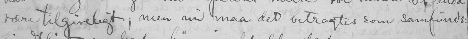være tilgiveligt; men nu maa det betragtes som samfunds-
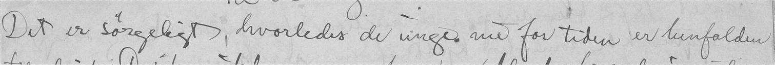Det er sørgeligt, hvorledes de unge med for tiden er henfalden
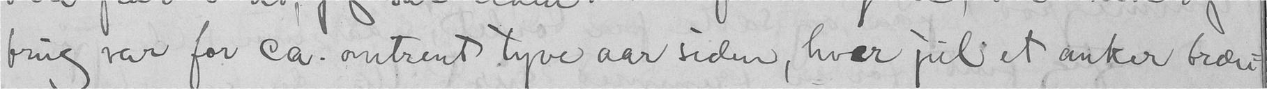brug var for ca. omtrent tyve aar siden, hver jul et anker bræn-
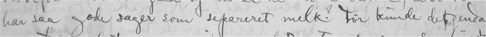har saa gode sager som separeret melk? Før kunde det enda
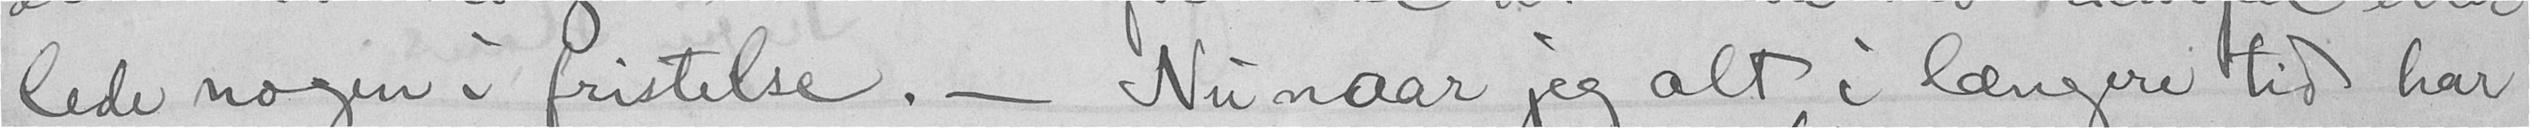lede nogen i fristelse. - Nu naar jeg alt i længere tid har
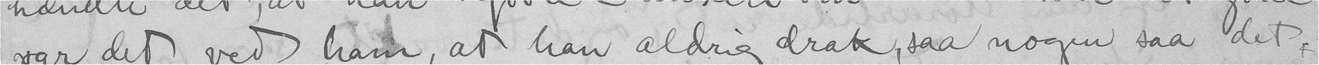var det ved ham, at han aldrig drak, saa nogen saa det,
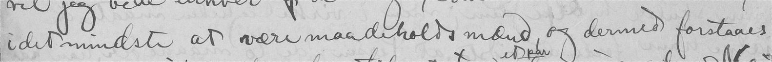idetmindste at være maadeholds mænd og dermed forstaaes
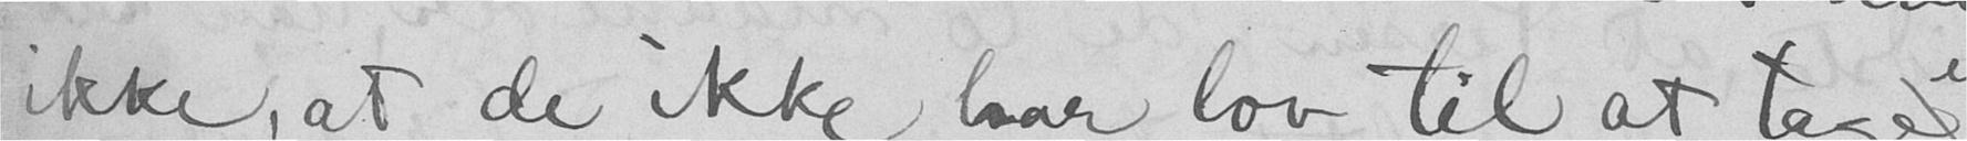ikke, at de ikke har lov til at tage
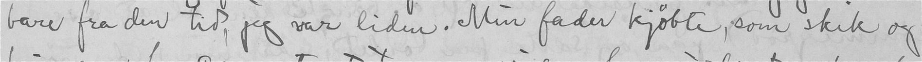bare fra den tid, jeg var liden. Min fader kjøbte, som skik og
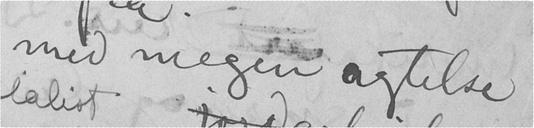med megen agtelse
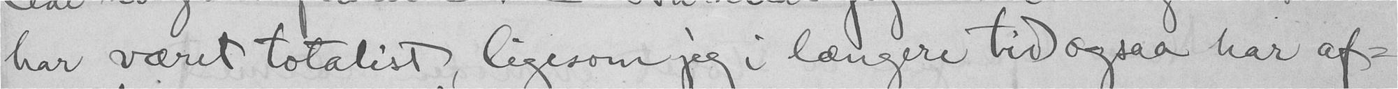har været totalist, ligesom jeg i længere tid ogsaa har af-
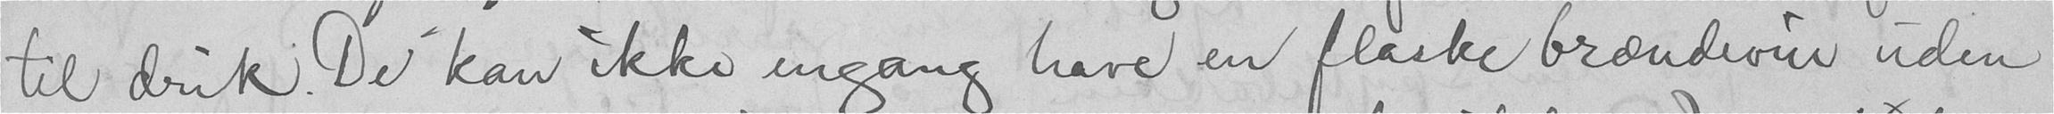til drik. De kan ikke engang have en flaske brændevin uden
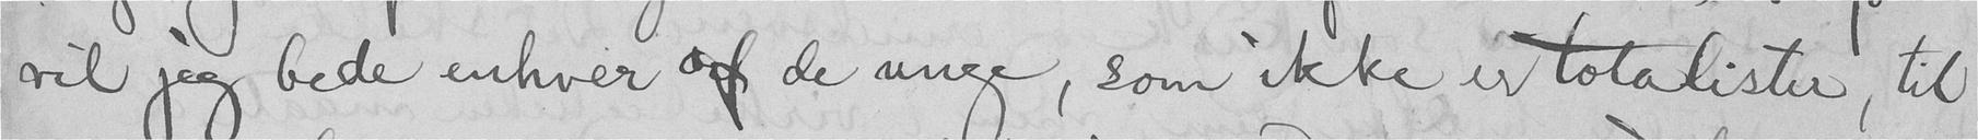vil jeg bede enhver af de unge, som ikke er totalister, til
